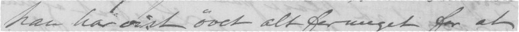han har vist øvet alt formeget for at
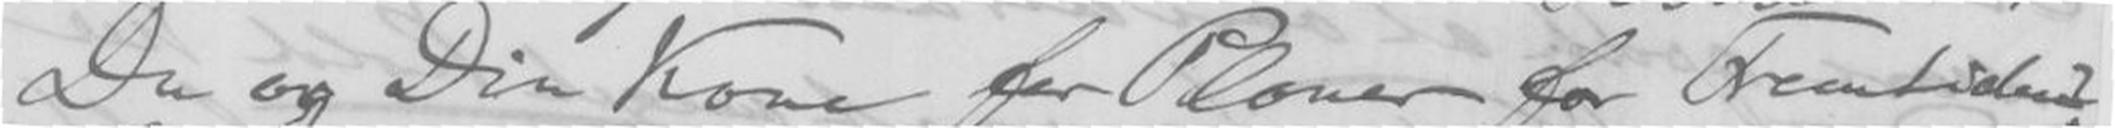Du og Din Kone for Planer for Fremtiden.
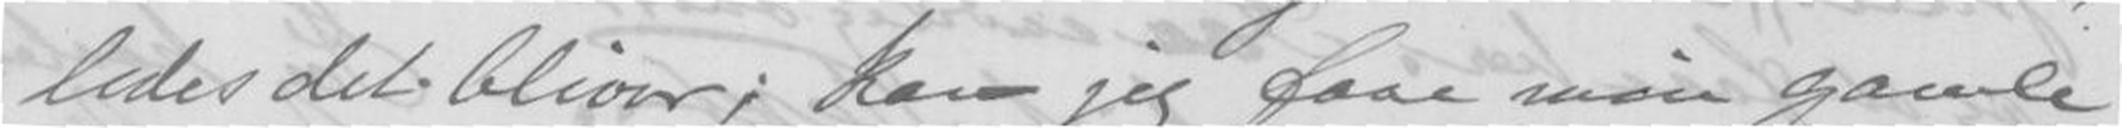ledes det bliver; kan jeg faae min gamle
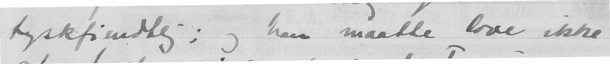tyskfiendtlig; og han maatte love ikke
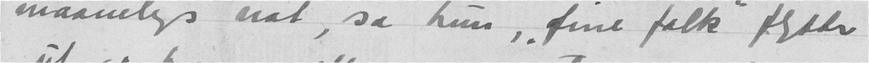maanelys nat, sa hun, "fine folk" flytter
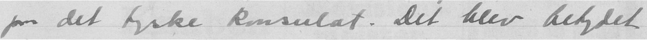paa det tyske konsulat. Det blev betydet
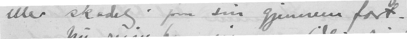eller skadelig paa sin gjennemfart.
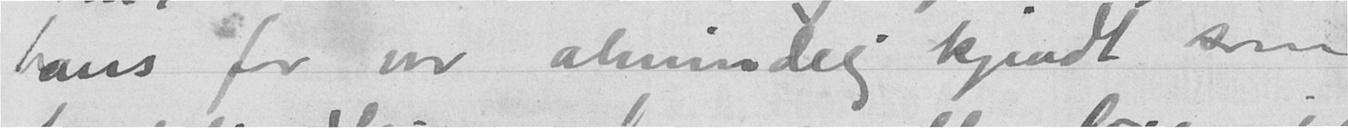hans far var almindelig kjendt som
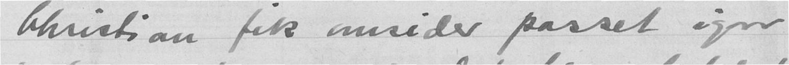Christian fik omsider passet igaar
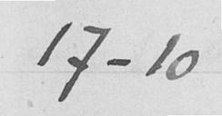17-10
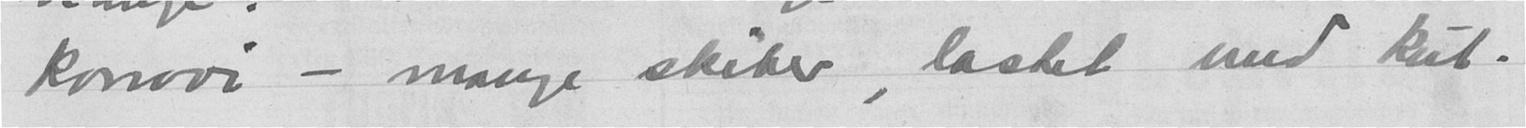konvoi - mange skiber lastet med kul.
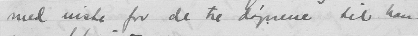med niste for de tre døgnene til han
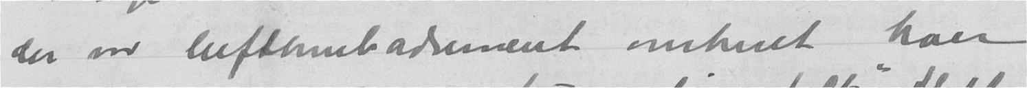der var luftbombardement omtrent hver
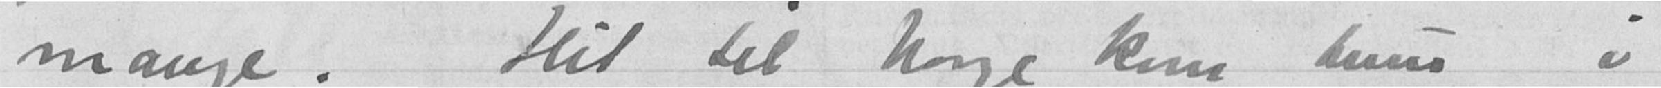mange. Hit til Norge kom hun i
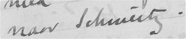naar Schweitz.
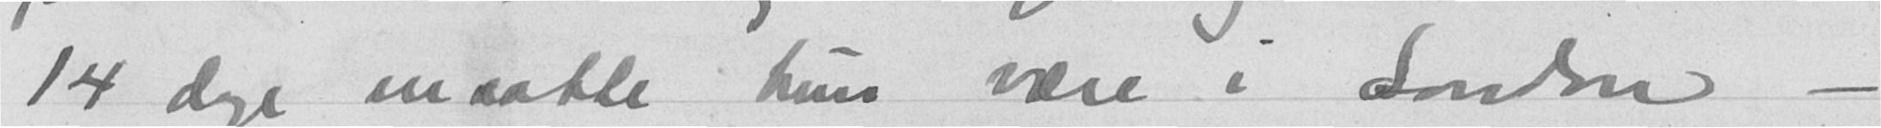14 dage maatte hun være i London -
